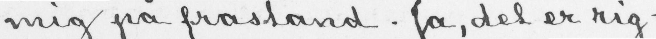mig på frastand. Ja, det er rig-
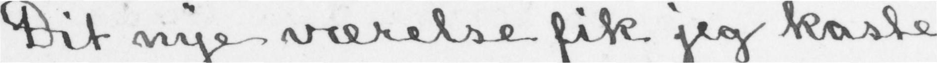Dit nye værelse fik jeg kaste
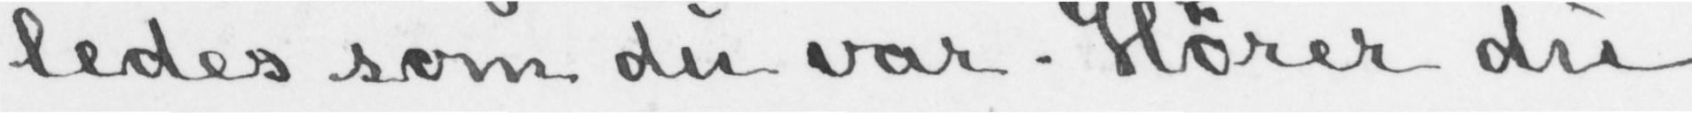ledes som du var. Hører du
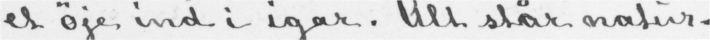et øje ind i igår. Alt står natur-
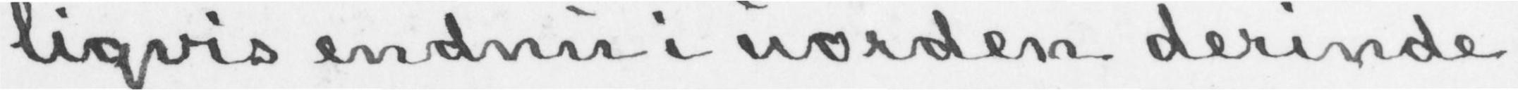ligvis endnu i uorden derinde.
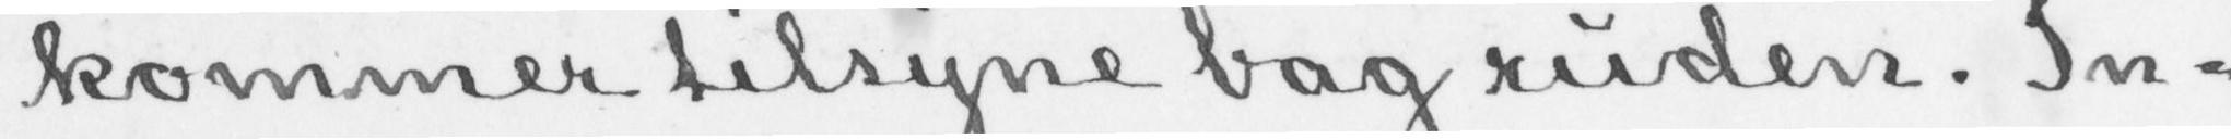kommer tilsyne bag ruden. In-
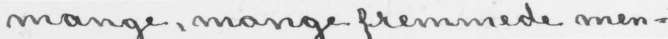mange, mange fremmede men-
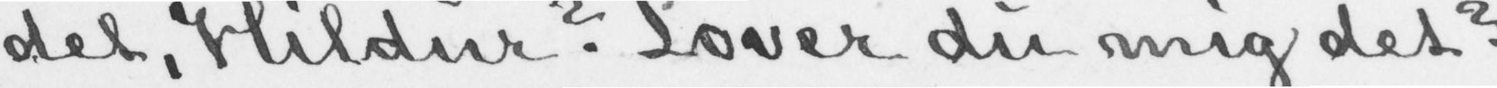det, Hildur? Lover du mig det?
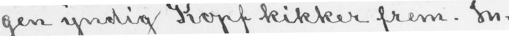gen yndig Kopf kikker frem. In-
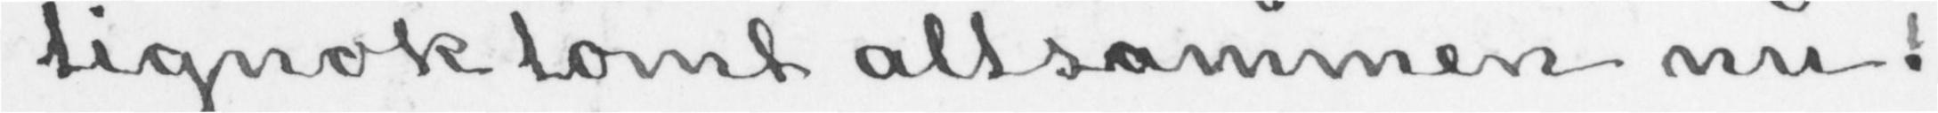tignok tomt altsammen nu!
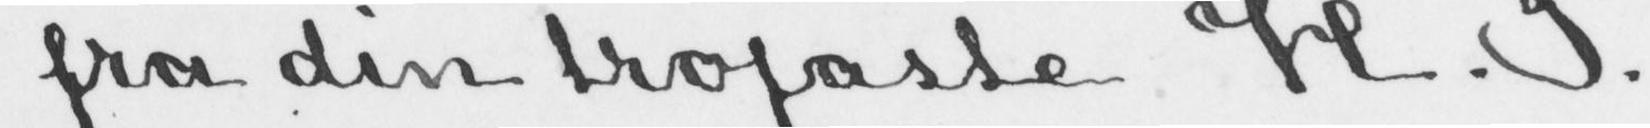fra din trofaste H. I.
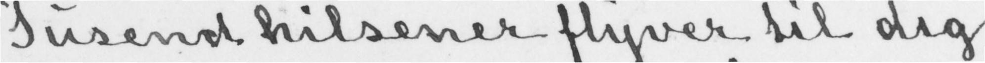Tusend hilsener flyver til dig
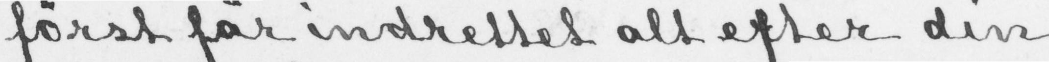først får indrettet alt efter din
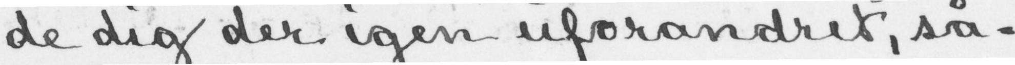de dig der igen uforandret, så-
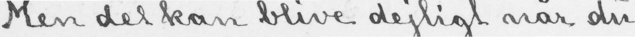Men det kan blive dejligt når du
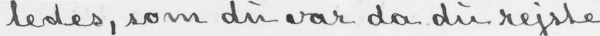ledes, som du var da du rejste
